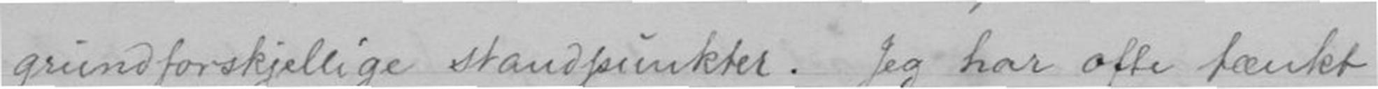grundforskjellige standpunkter. Jeg har ofte tænkt
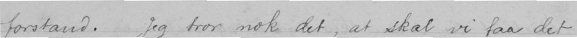forstand. Jeg tror nok det, at skal vi faa det
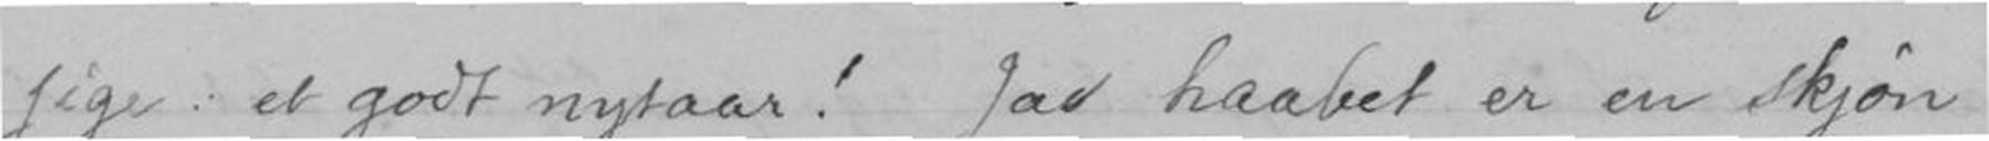sige et godt nytaar! Gav haabet er en skjøn
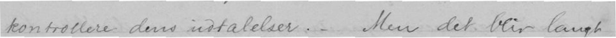kontrollere dens udtalelser. - Men det blir langt
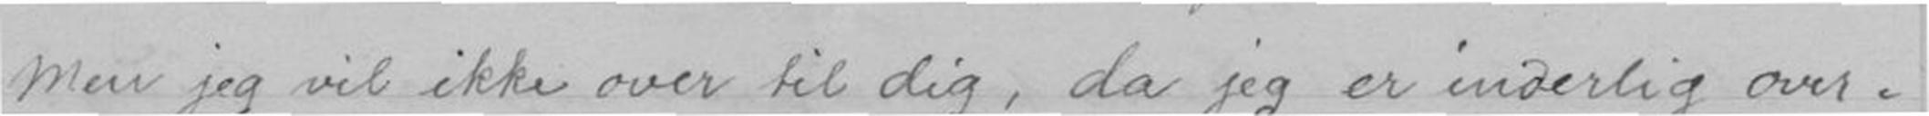Men jeg vil ikke over til dig, da jeg er inderlig over-
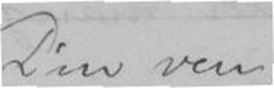Din ven
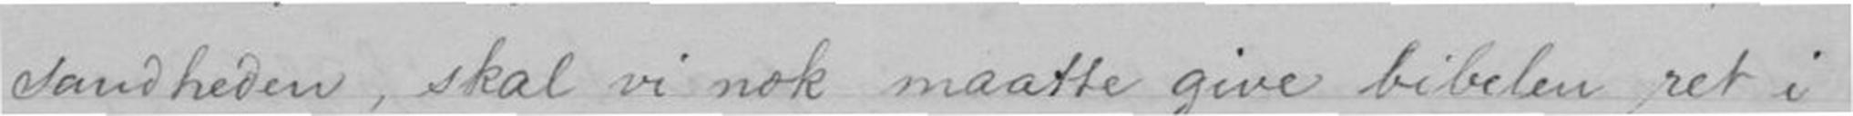sandheden, skal vi nok maatte give bibelen ret i
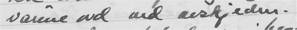varme ord ved avskjeden.
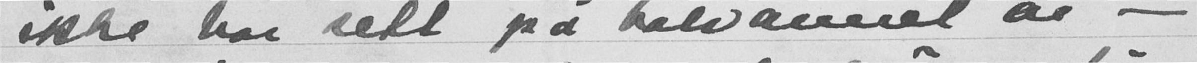ikke har sett på halvannet år -
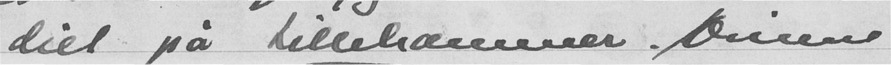diet på Lillehammer. Dimm
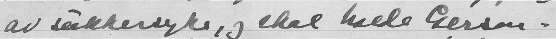av sukkersyke, og skal holde Gerson-
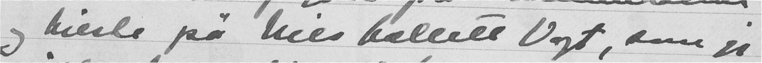og hilste på Nils Kollett Vogt, som jeg
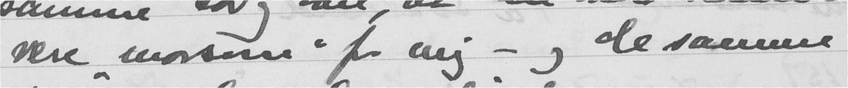være "morsom" for mig - og de samme
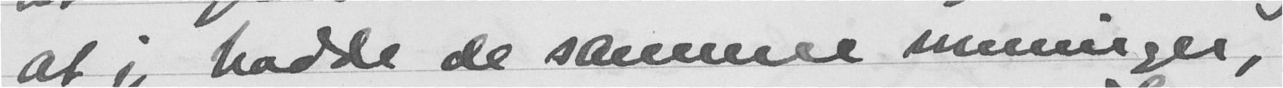at jeg hadde de samme meninger,
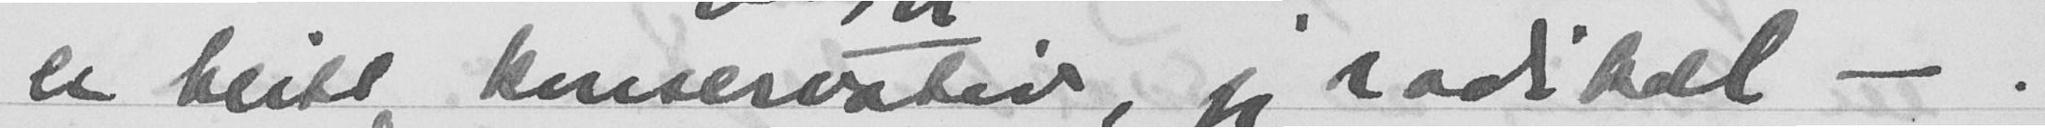er blitt konservativ, jeg radikal - .
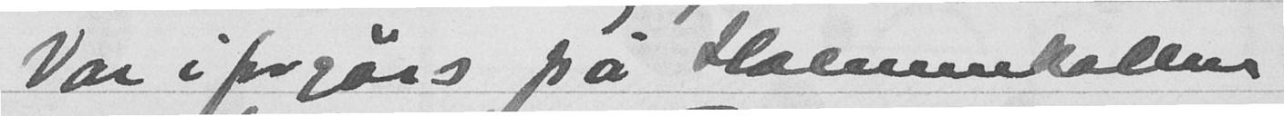Var i forgårs pa Holmenkollen
